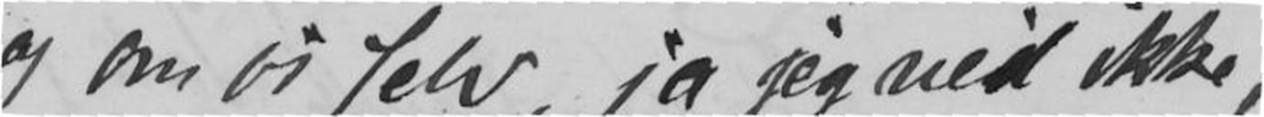og om os selv, ja jeg ved ikke,
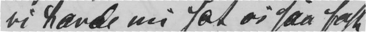vi havde nu sæt os saa fast
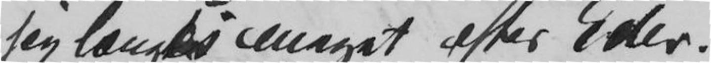jeg længtes meget efter Eder.
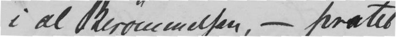i al Berømmelsen, - pratet
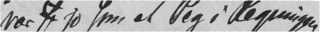var s jo som et Peg i Regningen,
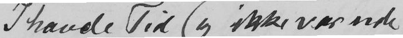I havde Tid og ikke var ude
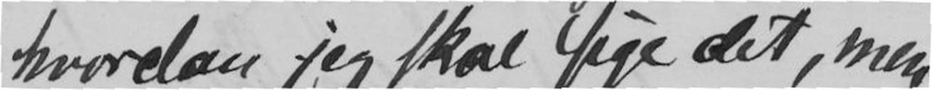hvordan jeg skal sige det, men
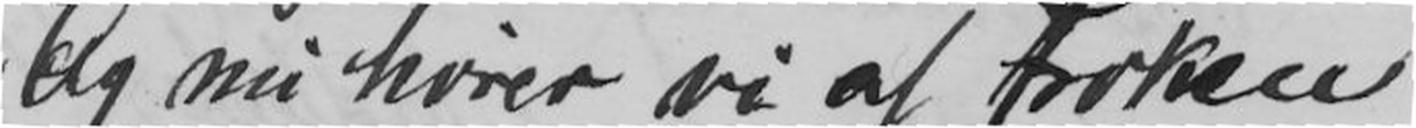Og nu hører vi af Frøken
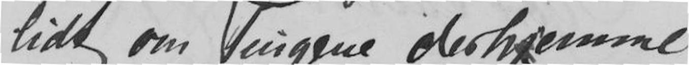lidt om Tingene der hjemme
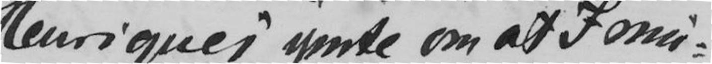Henriques ymte om at I mu-
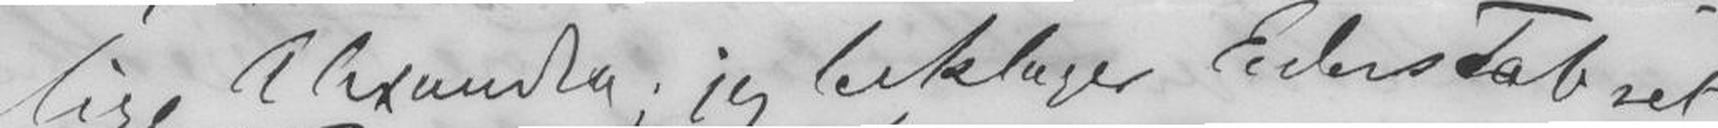lige Alexandra; jeg beklager Eders Tab ret
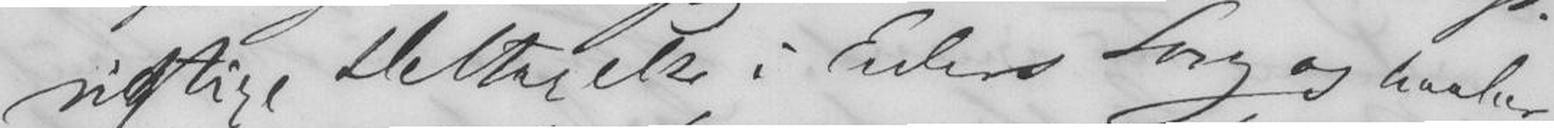rigtige Deltagelse i Eders Sorg og haaber,
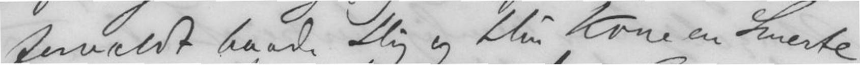forvoldt baade Dig og Din kone en Smerte
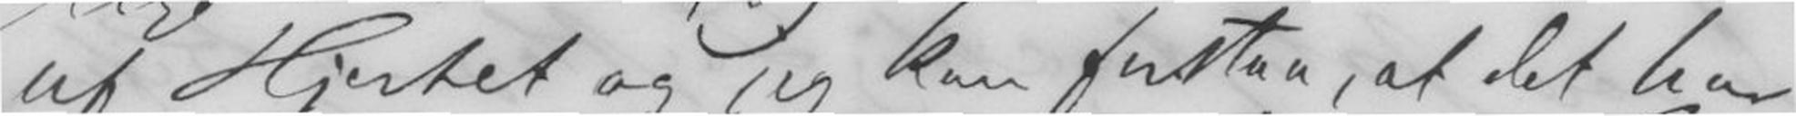af Hjertet og jeg kan forstaa, at det har
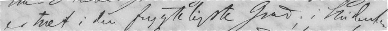er træt i den frygteligste Grad; i Studenter-
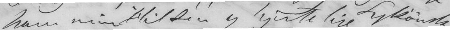ham min Hilsen og hjertelige Lykønsk
